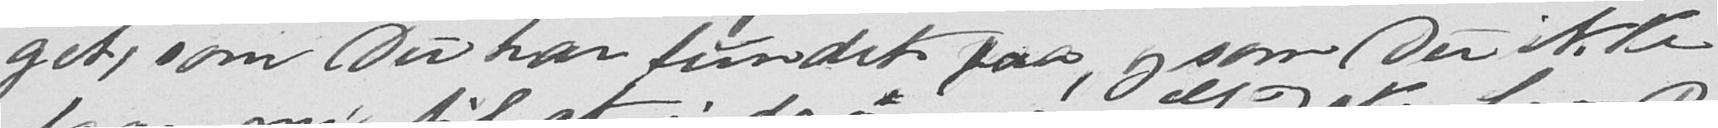get, som Du har fundet faa, og som Du ikke
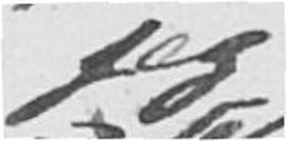jeg
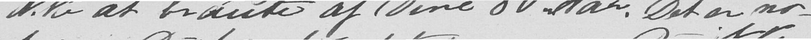ikke at braute af Dine 80 Aar. Det er no-
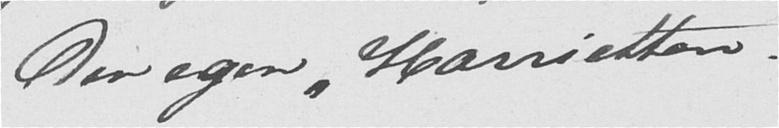Din egen, Harrietten
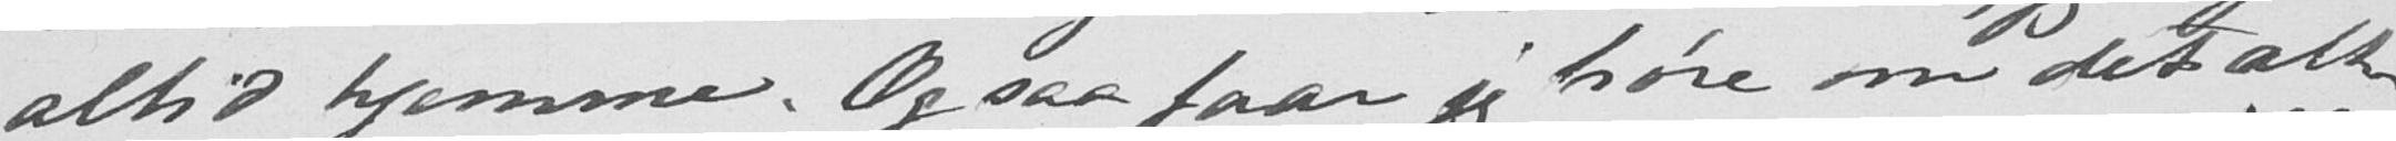altid hjemme. Og saa faar jeg høre om det alt
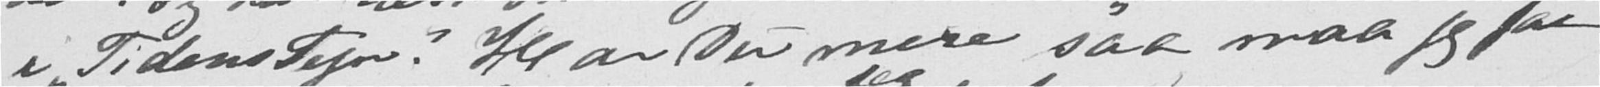i "Tidens tegn." Har Du mere saa maa jeg faa
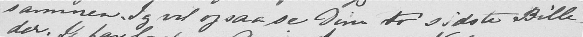sammen. Jeg vil ogsaa se Dine tr sidste Bille-
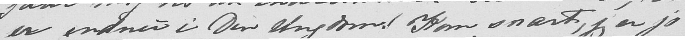er endnu i Din Ungdom! Kom snart, jeg er jo
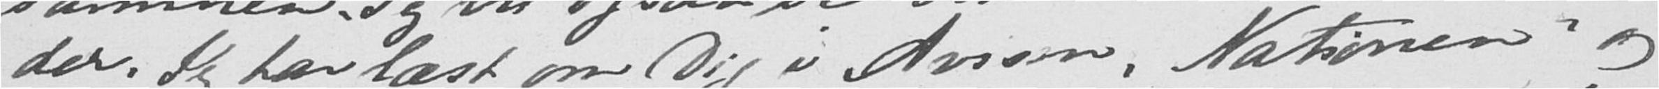der. Jeg har læst om Dig i Avisen, "Nationen" og
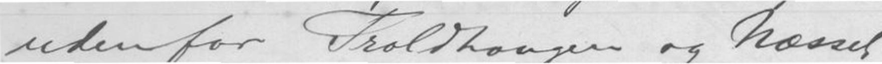udenfor Troldhaugen og Næsset
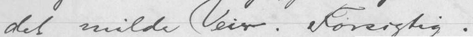det milde Veir. Forsigtig.
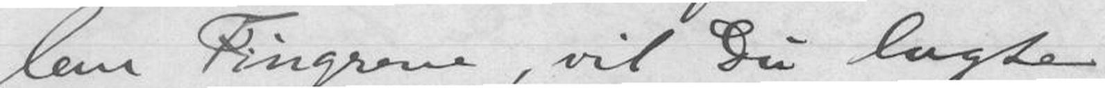lem Fingrene, vil Du lugte
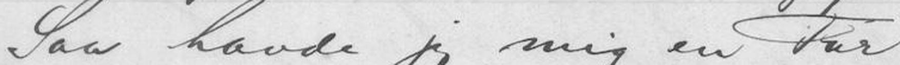Saa havde jeg mig en Tur
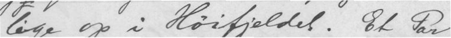lige op i Høifjeldet. Et Par
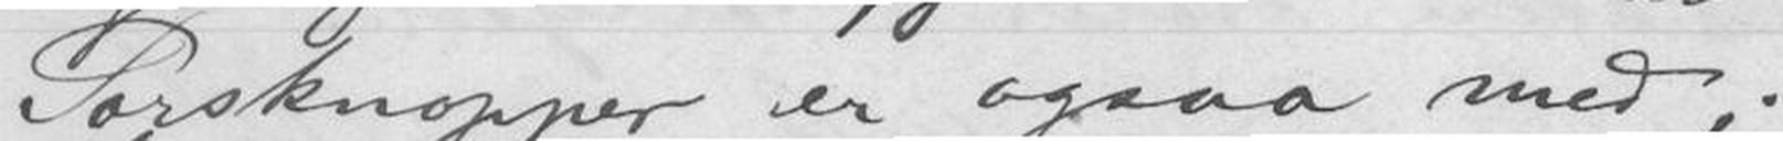Porsknopper er ogsaa med;
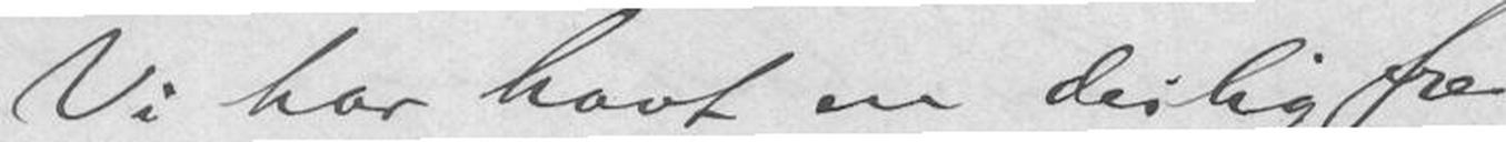Vi har havt en deilig fre-
